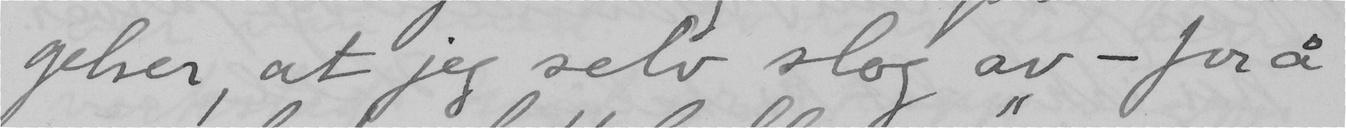gelser, at jeg selv slog av – for å
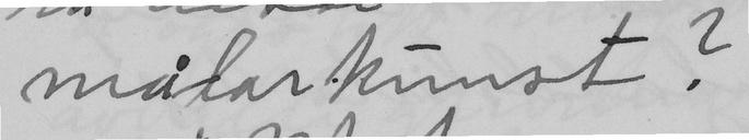malarkunst?
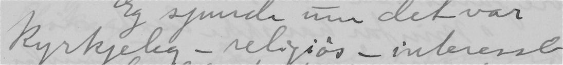kyrkjeleg – religiös – interesse
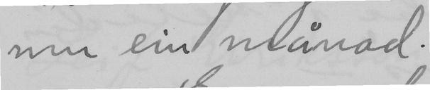um ein månad.
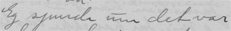Eg sjunde um det var
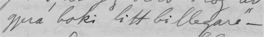gjera boki litt billegare" –
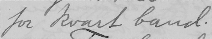for kvart band.
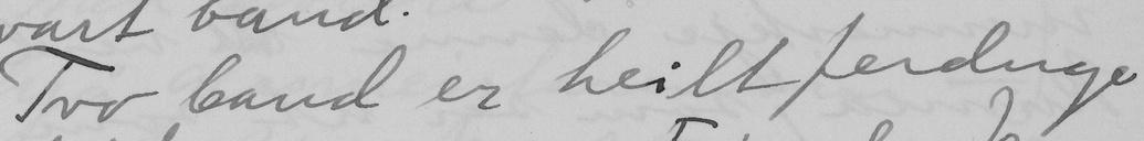Tvo band er heilt ferdige
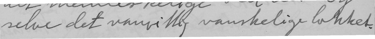selve det vanvittig vanskelige lokket.
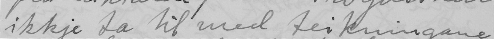ikkje ta til med teikningane
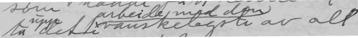ta upp dette arbeide med den vanskelegste av all
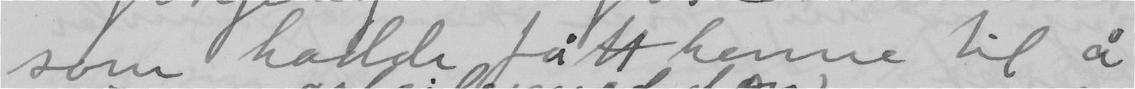som hadde fått henne til å
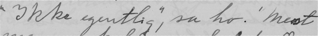"Ikke egentlig", sa ho. Mest
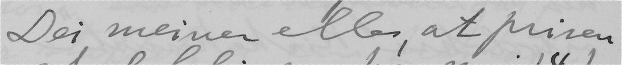Dei meiner elles at prisen
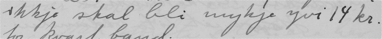ikkje skal bli mykje yvi 14 kr.
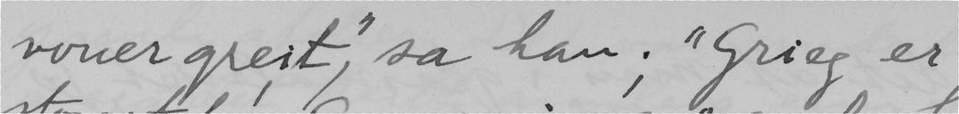voner greit", sa han; "Grieg er
